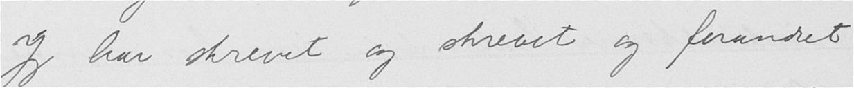Jeg har skrevet og skrevet og forandret
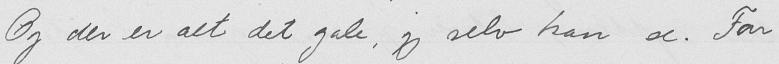Og der er alt det gale, jeg selv kan se. For
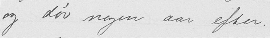og dør nogen aar efter.
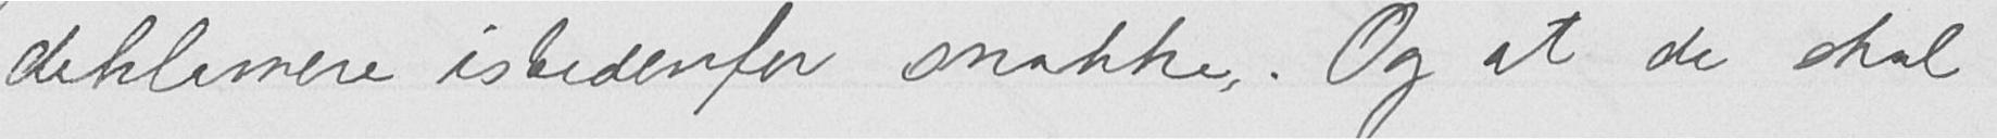deklamere istedenfor snakke. Og at de skal
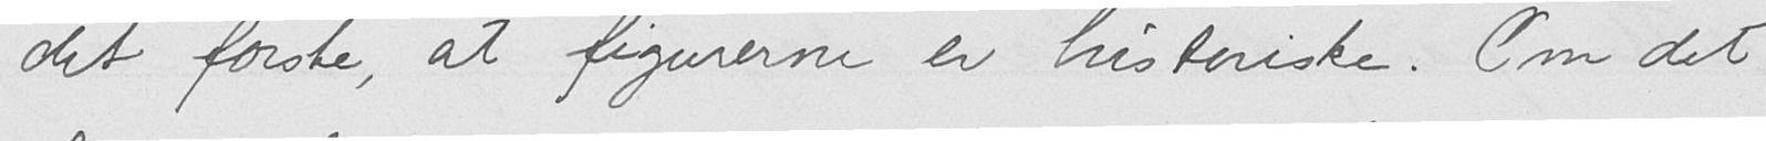det første, at figurerne er historiske. Om det
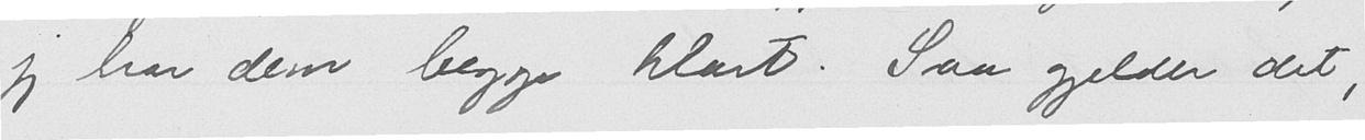jeg har dem begge klart. Saa gjelder det,
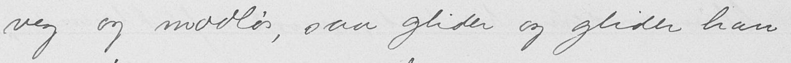veg og modløs, saa glider og glider han
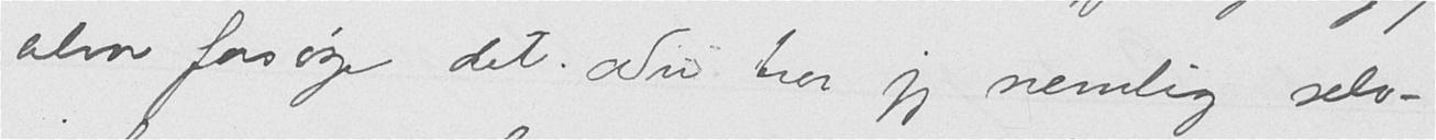alvor forsøge det. Nu tror jeg nemlig selv,
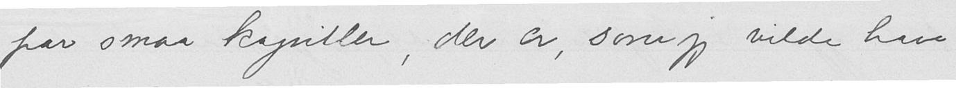par smaa kapitler, der er, som jeg vilde have
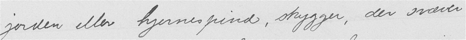jorden eller hjernespind, skygger, der svæver
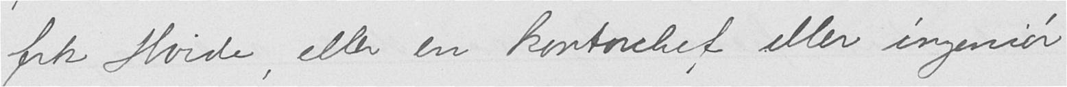frk Hvide, eller en kontorchef eller ingeniør
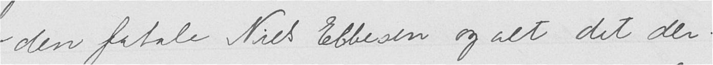- den fatale Niels Ebbesen og alt det der.
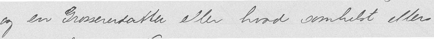og en Grossererdatter eller hvad somhelst ellers
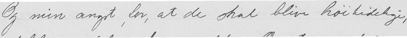Og min angst for, at de skal blive høitidelige,
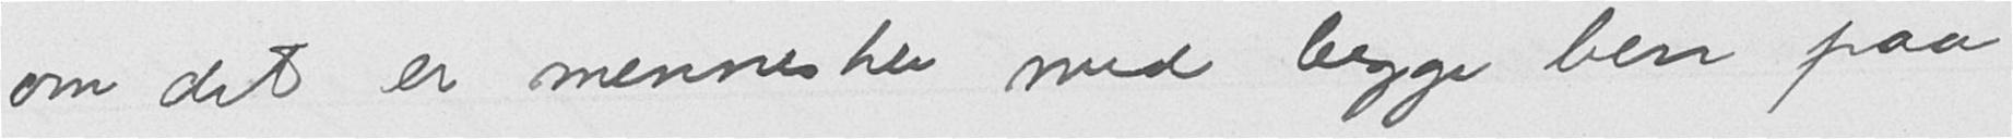om det er mennesker med begge ben paa
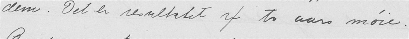dem. Det er resultatet af to aars møie.
